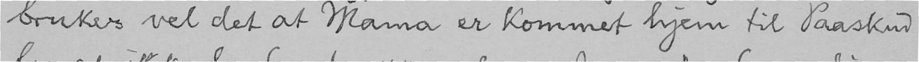bruker vel det at Mama er kommet hjem til Paaskud
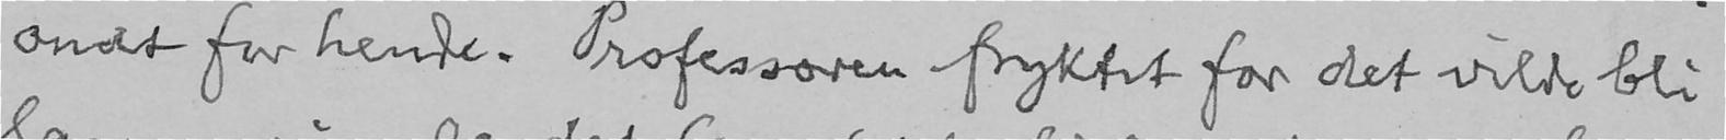ondt for hende. Professoren fryktet for det vilde bli
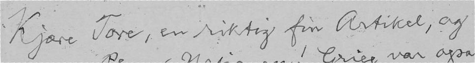Kjære Tore, en riktig fin Artikel, og
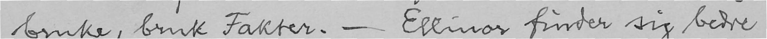bruke, bruk Fakter. - Ellinor finder sig bedre
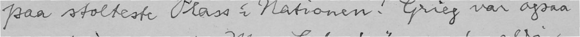paa stolteste Plass i Nationen! Grieg var ogsaa
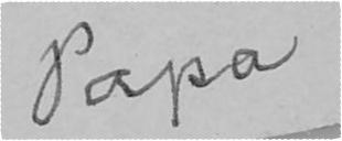Papa
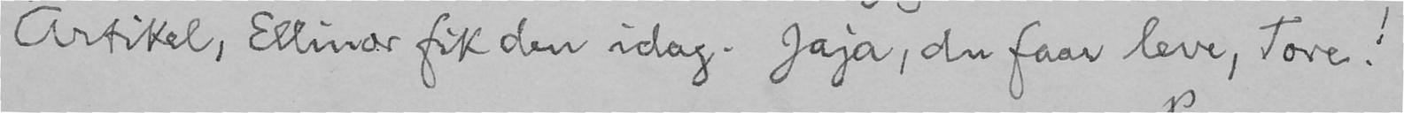Artikel, Ellinor fik den idag. Jaja, du faar leve, Tore!
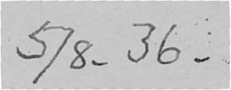5/8. 36.
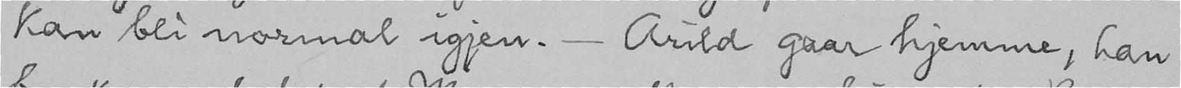kan bli normal igjen. - Arild gaar hjemme, han
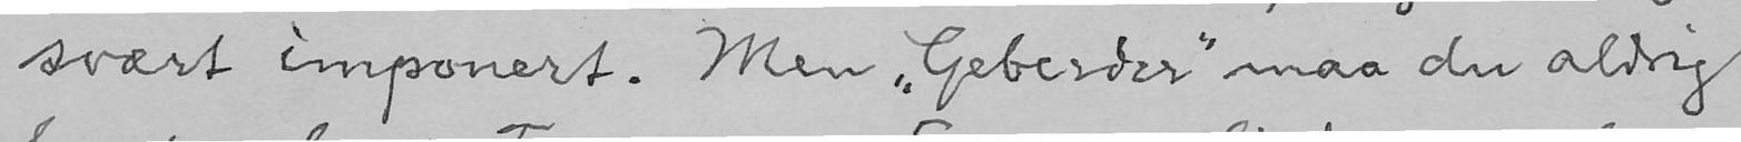svært imponert. Men "Geberder" maa du aldrig
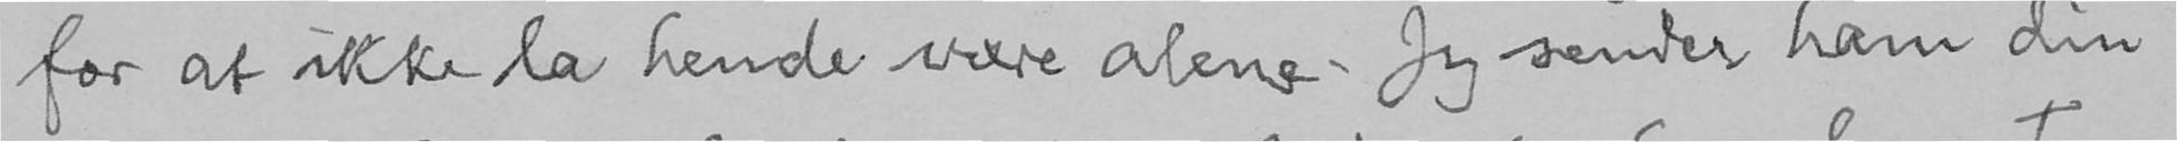for at ikke la hende være alene. Jeg sender ham din
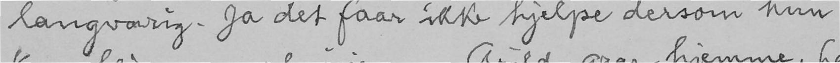langvarig. Ja det faar ikke hjelpe dersom hun
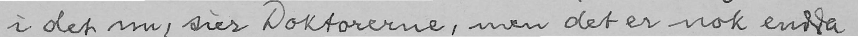i det nu, sier Doktorerne, men det er nok endda
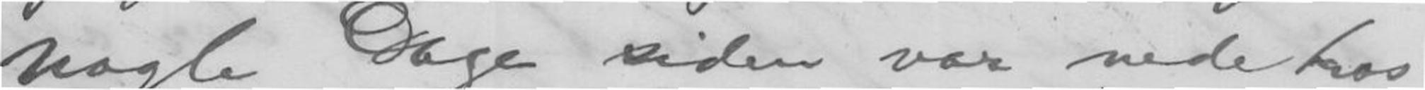nogle Dage siden var nede hos
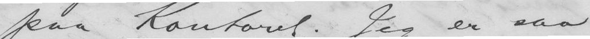paa Kontoret. Jeg er saa
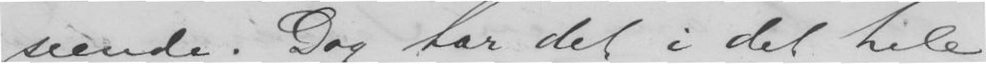seende. Dog har det i det hele
